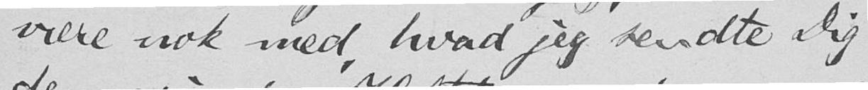være nok med, hvad jeg sendte Dig
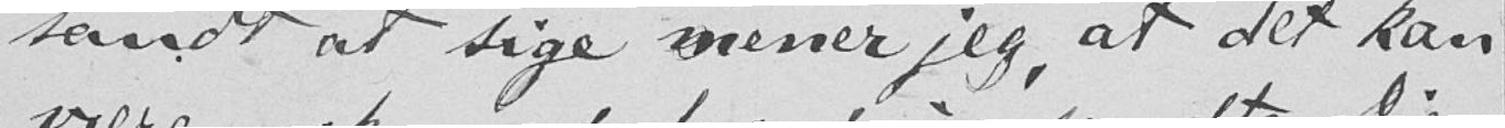sandt at sige mener jeg, at det kan
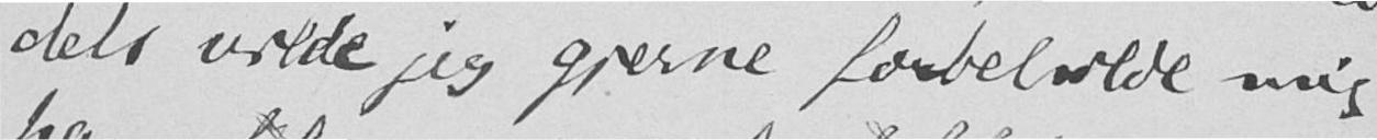dels vilde jeg gjerne forbeholde mig
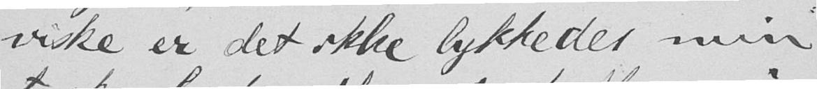viske er det ikke lykkedes min
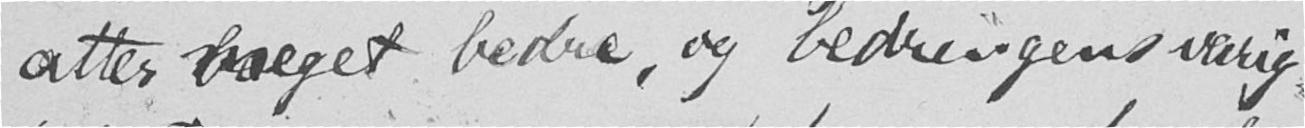atter meget bedre, og bedringens varig-
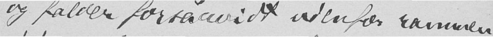og falder forsaavidt udenfor rammen,
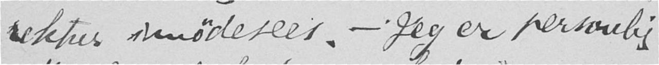rektur imødesees. - Jeg er personlig
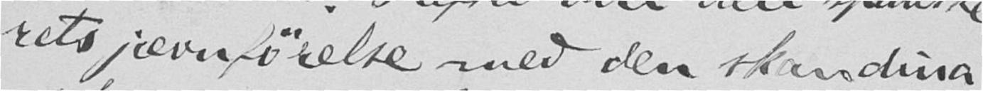rets jævnførelse med den skandina-
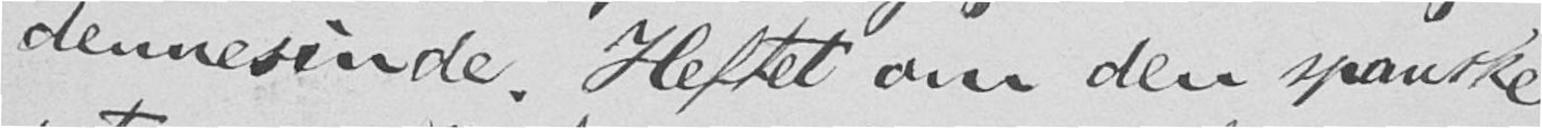dennesinde. Heftet om den spanske
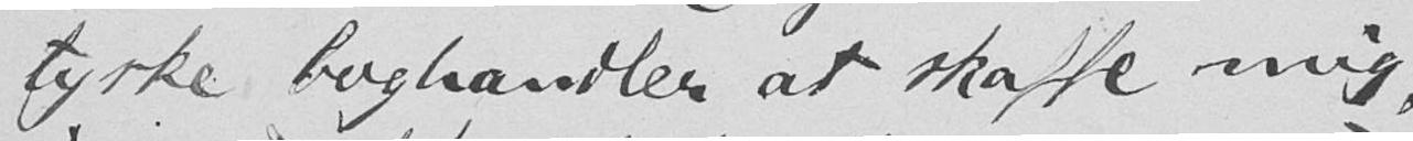tyske boghandler at skaffe mig,
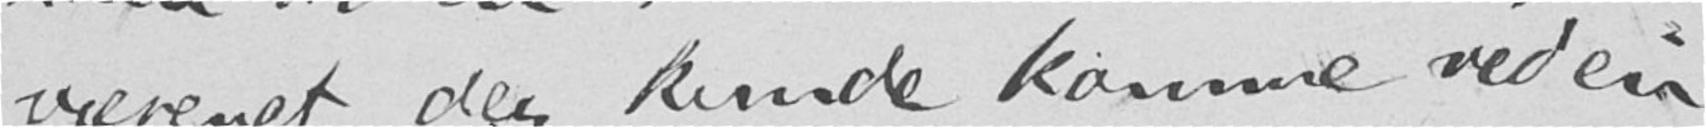væsenet, der kunde komme ved en
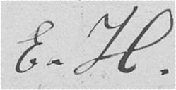E. H.
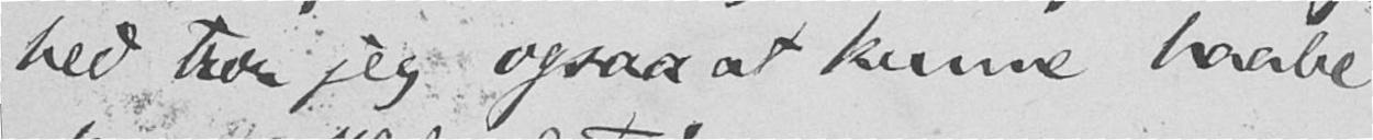hed tror jeg ogsaa at kunne haabe
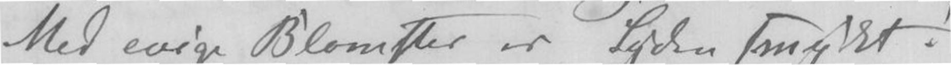Med evige Blomster er Syden smykt!
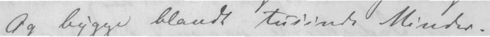Og bygge blandt tusinde Minder.
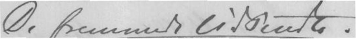De fremmede tidsendte.
